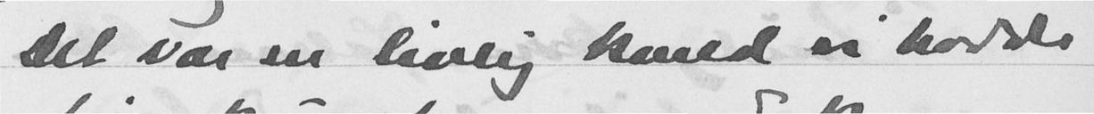Det var en livlig kveld vi hadde
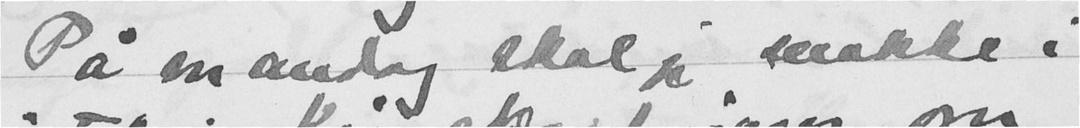På mandag skal jeg snakke i
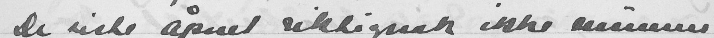De siste åpnet riktignok ikke munnen
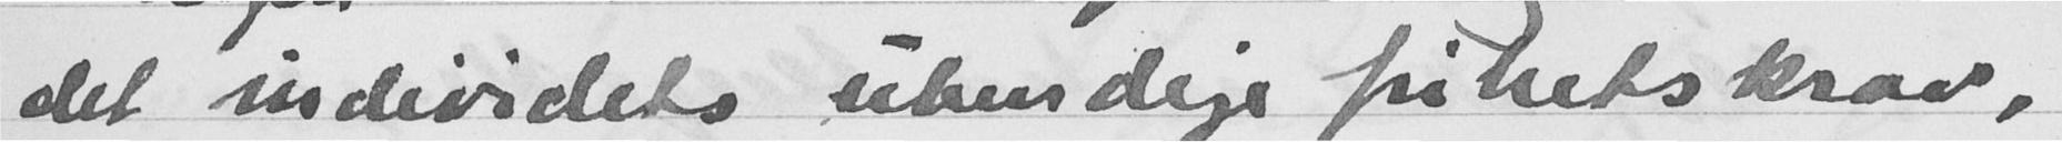det individets ubendige frihetsbehov,
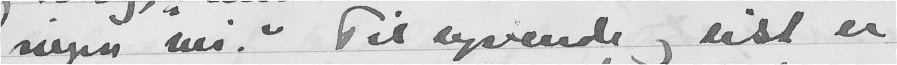ingen vei. Til syvende og sist er
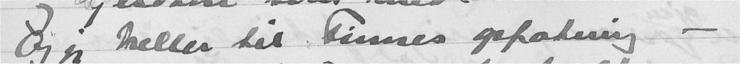Og jeg heller til Finnes opfatning -
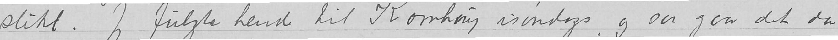slikt. Jeg fulgte hende til Kornhaug isøndags, og saa gaar det da
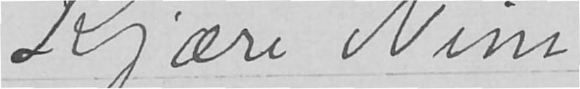Kjære Nini
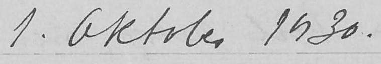1. Oktober 1930.
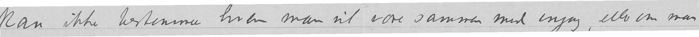er atten aar, men man kan ikke bestemme hvem man vil være sammen med engang, eller om man
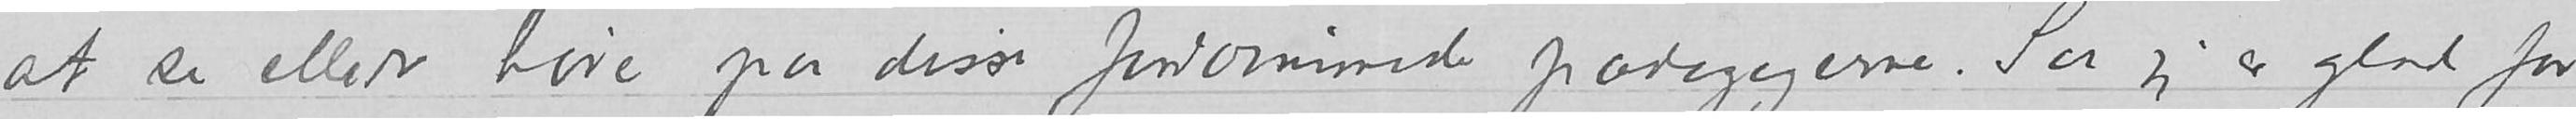at se eller høre paa disse fontovirrede pædagogerne  Saa jeg er glad for
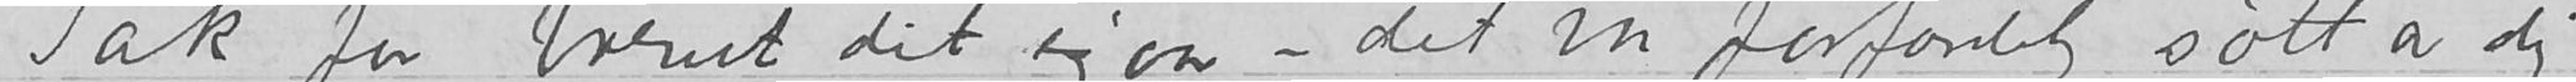Tak for brevet dit igaar - det var fordærdelig søtt av dig
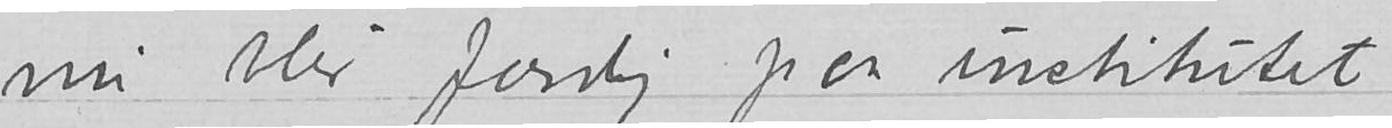blir færdig paa institutet.
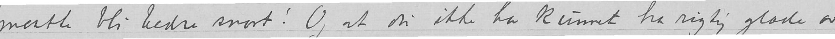maatte bli bedre snart! Og at du ikke har kunnet ha rigtig glæde av
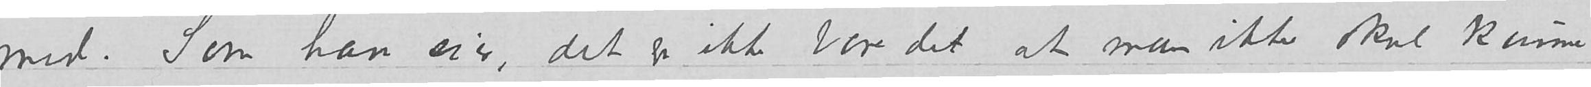med. Som han sier, det er ikke bare det at man ikke skal kunne
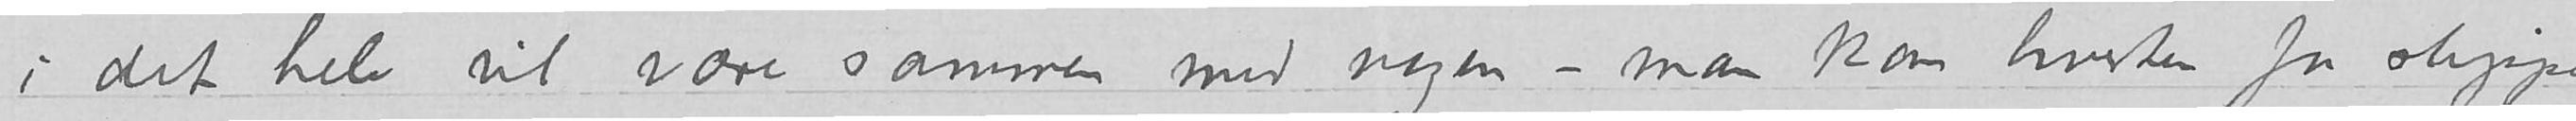i det hele vil være sammen med nogen – man kan hverken faa slippe
